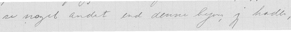se noget andet end denne byen, jeg hader,
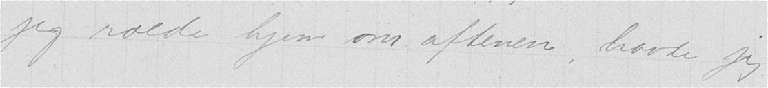jeg roede hjem om aftenen, havde jeg
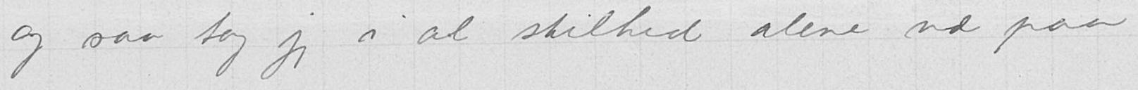og saa tog jeg i al stilhed alene ud paa
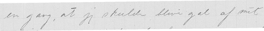en gang, at jeg skulde blive gal af mit
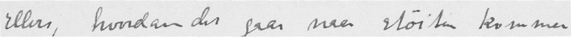Ellers, hvordan det gaar naar støiten kommer
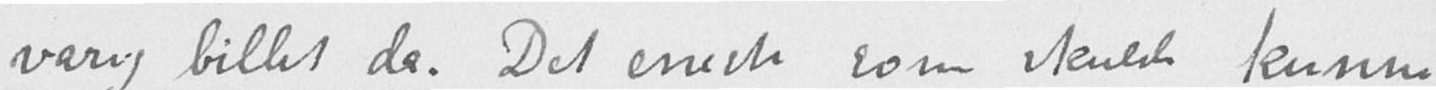varig billet da. Det eneste som skulde kunne
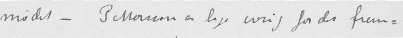mødet - Pettersson er lige ivrig for det frem-
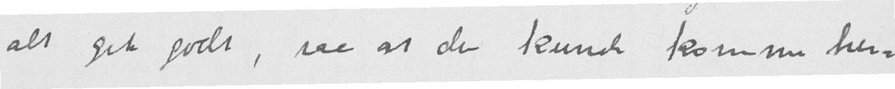alt gik godt, saa at du kunde komme her-
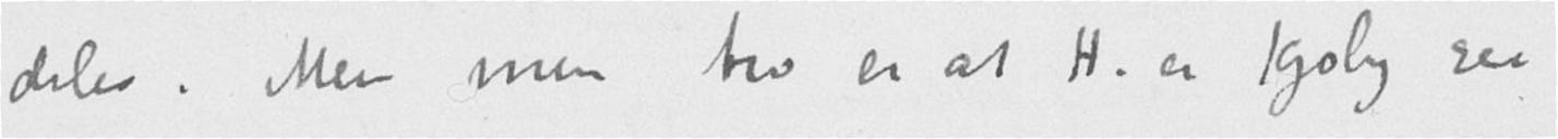deles. Men men tro er at H. er kjølig saa
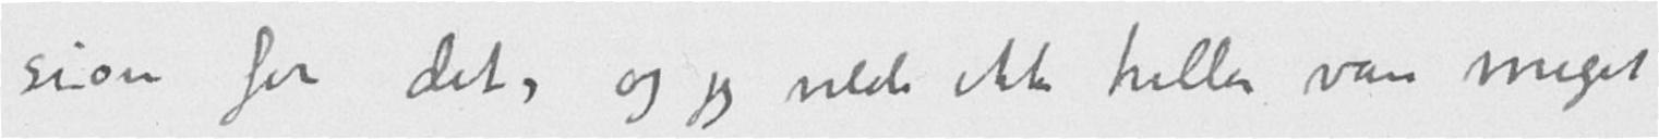sion for det, og jeg vilde ikke heller være meget
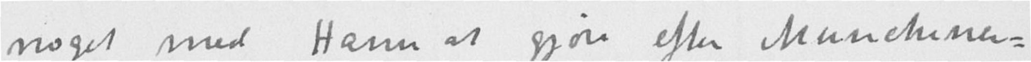noget med Hann at gjøre efter Munchener-
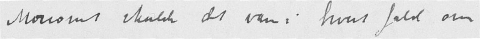Morsomt skulde det være i hvert fald om
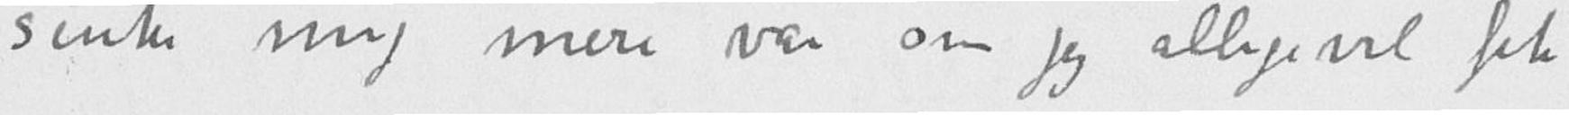sinke mig mere var om jeg alligevel fik
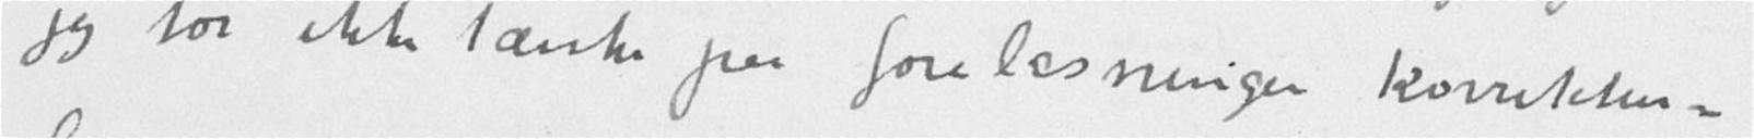jeg tør ikke tænke paa forelæsninger korrektur-
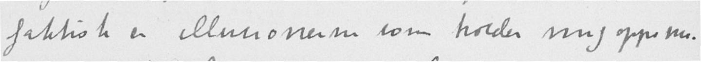faktisk er illusionerne som holder mig oppe nu.
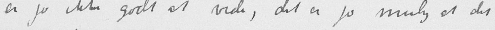er jo ikke godt at vide, det er jo mulig at det
